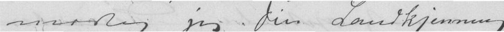modtog jeg Din Landkjenning,
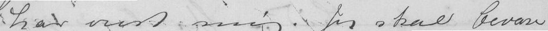har vist mig. Jeg skal bevare
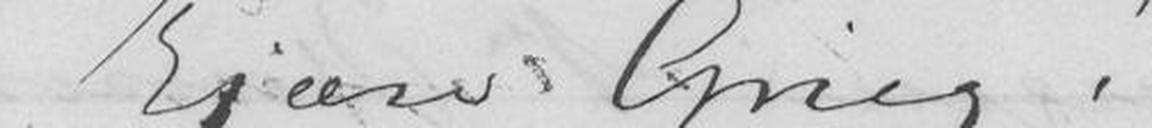Kjære Grieg!
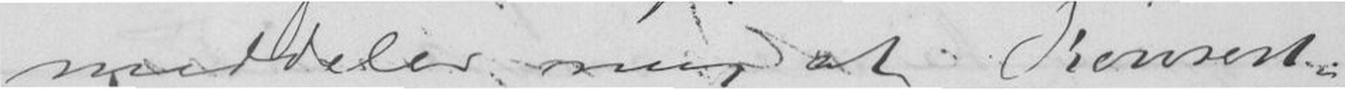meddeler mig at Konsert-
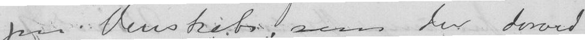paa Venskab, som Du derved
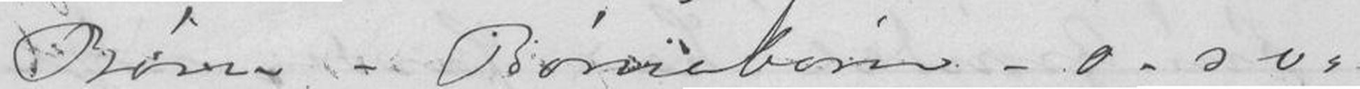Børn - Børnebørn - o. s. v.
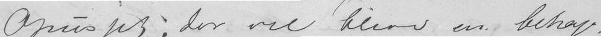Opusset; der vil blive en behage-
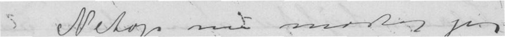Netop nu modtog jeg
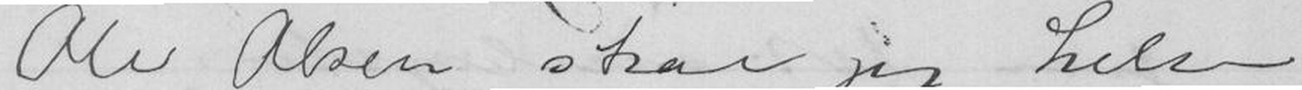Ole Olsen skal jeg hilse
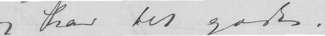og har det godt.
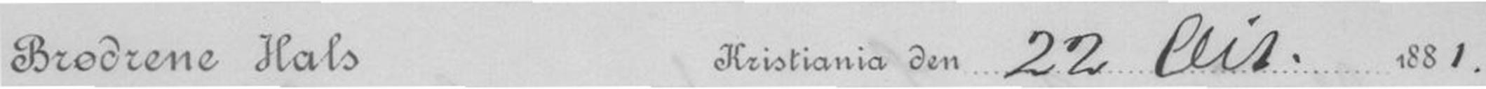Brødrene Hals Kristiania den 22 Oct 1881.
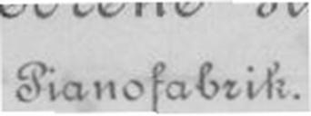Pianofabrik.
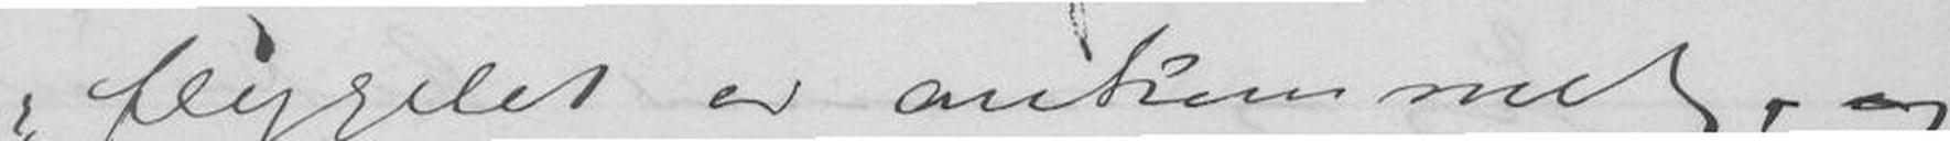flygelet er ankommet, og
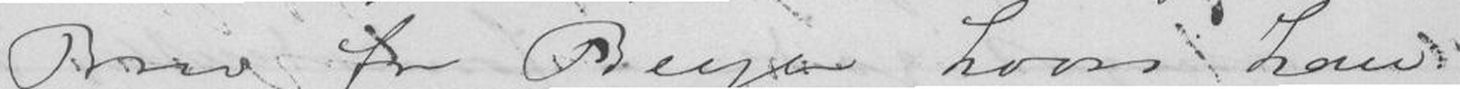Brev fra Bergen hvori han
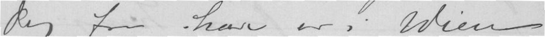Dig fra han er i Wien
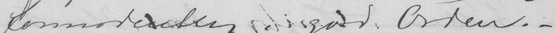formodentlig i god Orden. -
